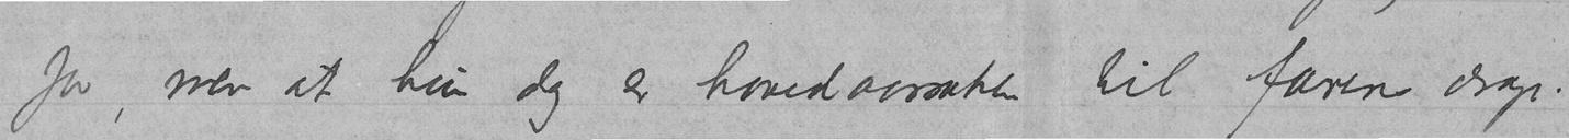for, men at hun dog er hovedaarsaken til farens drap.
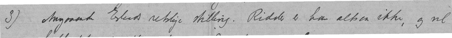3) Angaaende Erlends retslige stilling. Ridder er han altsaa ikke, og vel
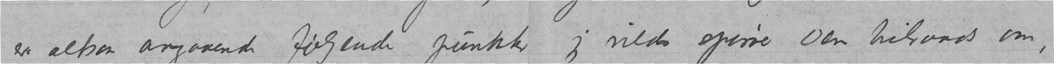er altsaa angaaende følgende punkter jeg vilde spørre Dem tilraads om,
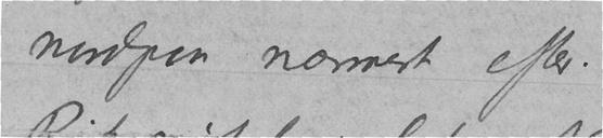nordpaa nærmest efter.
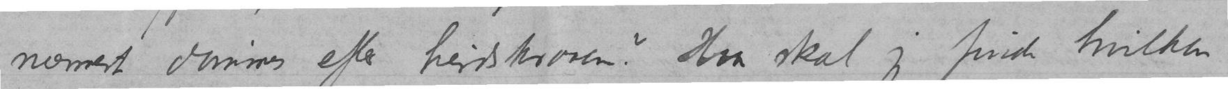nærmest dømmes efter hirdskraaen? Hvor skal jeg finde hvilken
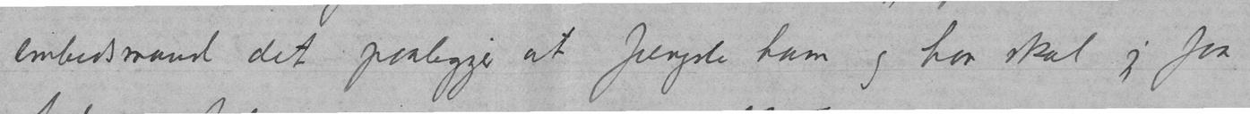embedsmand det paaligger at fengsle ham og hvor skal jeg faa
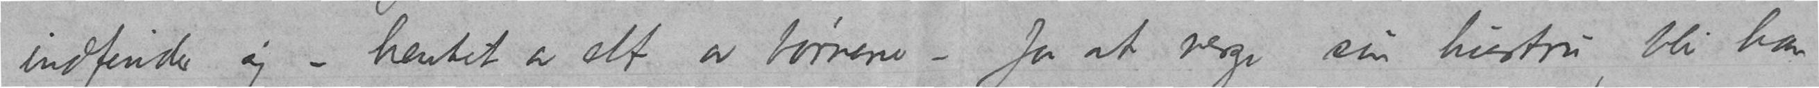indfinder sig – hentet av ett av børnene – for at verge sin hustru, blir han
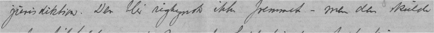jurisdiktion. Den blir rigtignok ikke fremmet – men den skulde
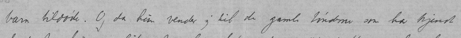barn tildøde. Og da hun vender sig til de gamle bønderne som har kjendt
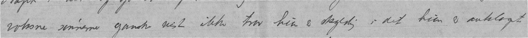voksne sønnerne ganske vist ikke tror hun er skyldig i det hun er anklaget
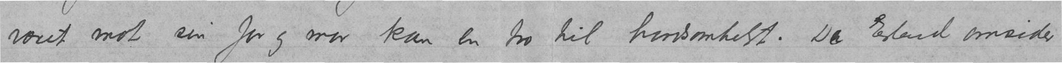været mot sin far og mor kan en tro til hvadsomhelst. Da Erlend omsider
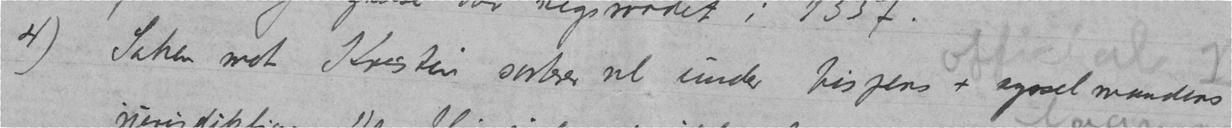4) Saken mot Kristin sorterer vel under bispens + sysselmandens
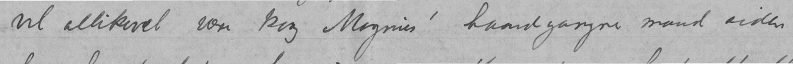vel allikevel være kong Magnus' haandgangne mand siden
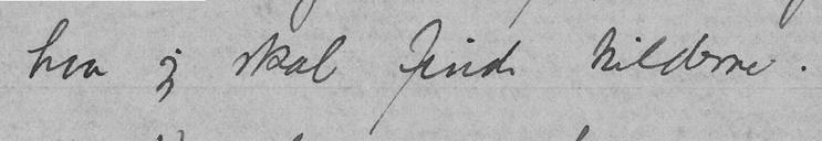hvor jeg skal finde kilderne.
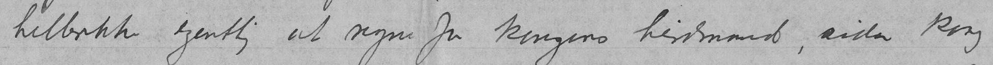hellerikke egentlig at regne for kongens hirdmand, siden kong
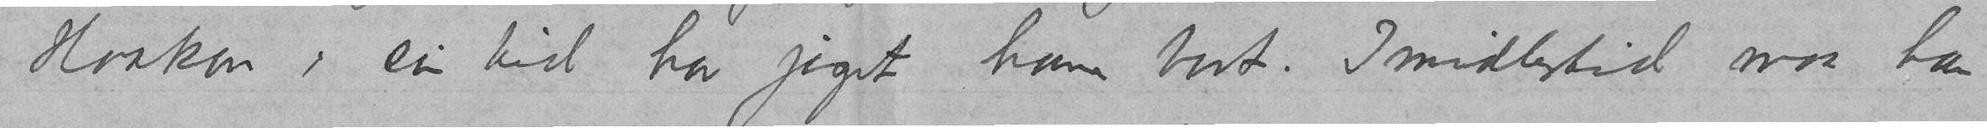Haakon i sin tid har jaget ham bort. Imidlertid maa han
